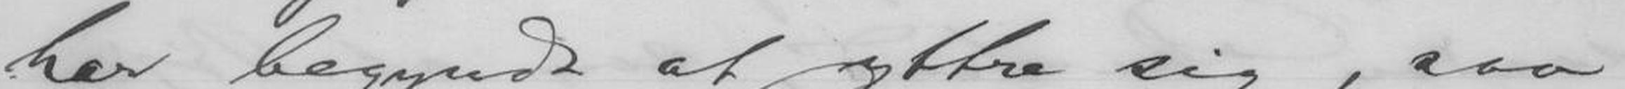har begyndt at yttre sig, saa
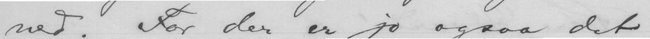ned. For der er jo ogsaa det
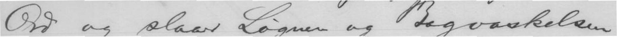Ord og slaar Løgnen og Bagvaskelsen
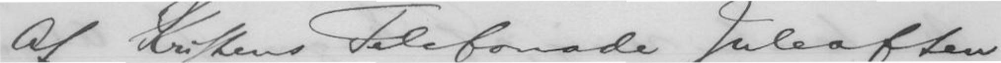Af Kristens Telefonade Juleaften
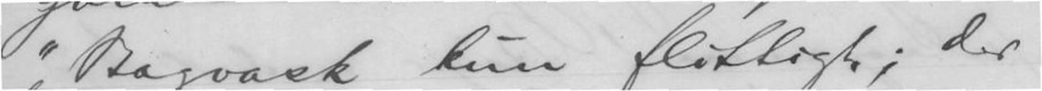"Bagvask kun flittigt; der
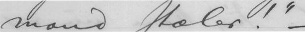mand stæler!"
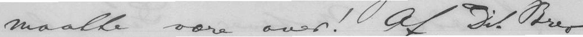maatte være over! Af Dit Brev
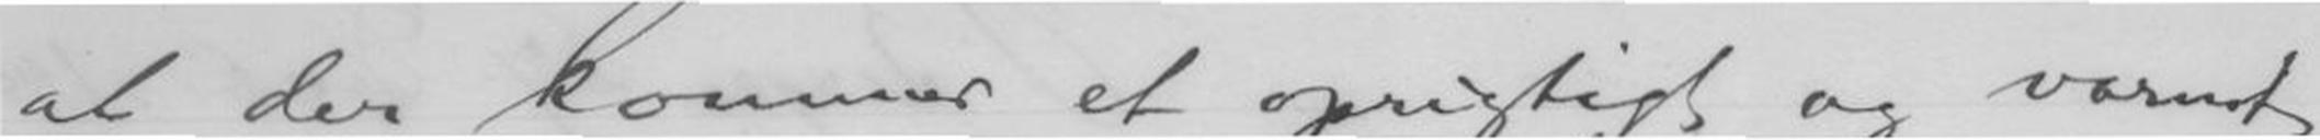at der kommer et oprigtigt og varmt
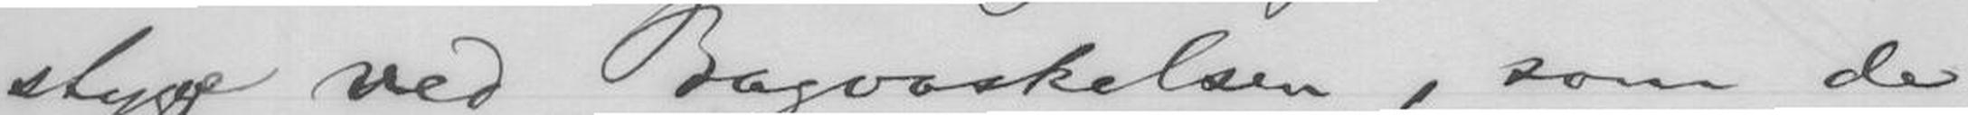stygge ved Bagvaskelsen, som de
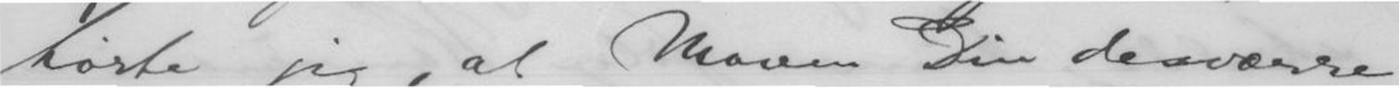hørte jeg, at Maven Din desværre
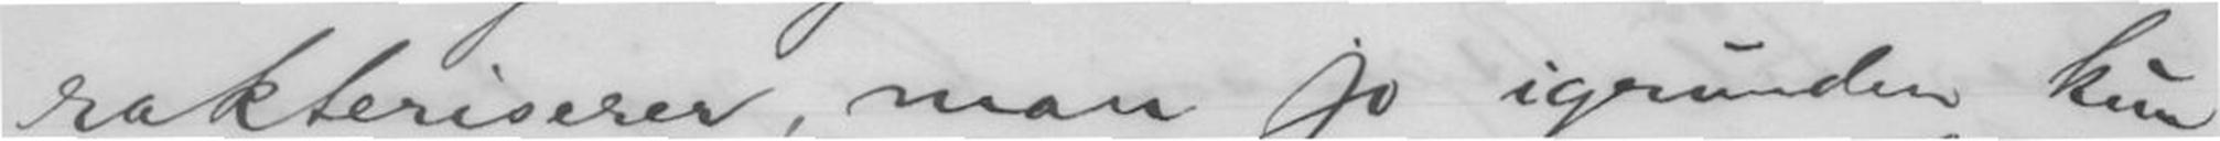rakteriserer, man jo igrunden kun
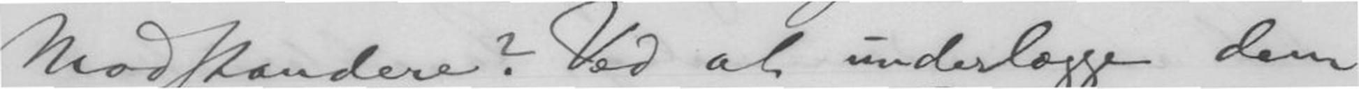Modstandere? Ved at underlægge dem
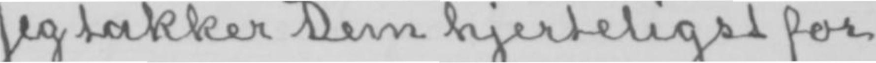Jeg takker Dem hjerteligst for
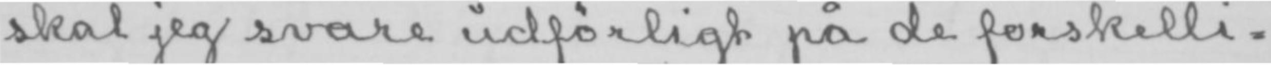skal jeg svare udførligt på de forskelli-
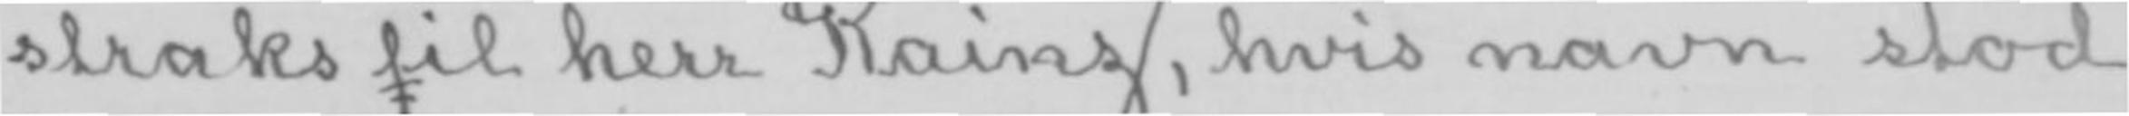straks ftil herr Kainz, hvis navn stod
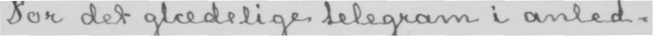For det glædelige telegram i anled-
